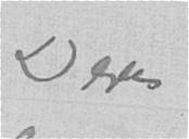Deres
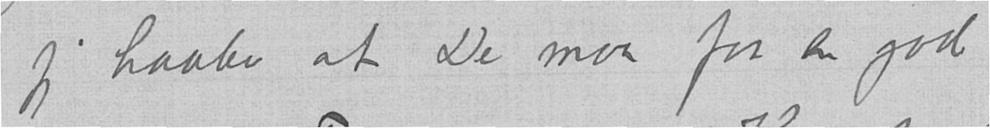jeg haabe at De maa faa en god
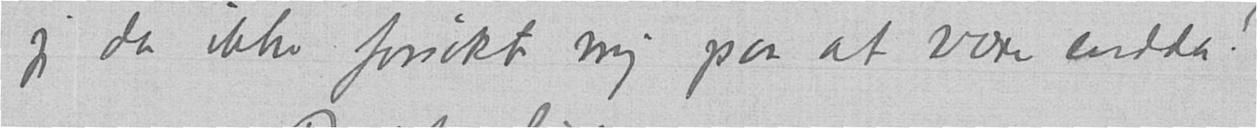jeg da ikke forsøkt mig paa at være endda.
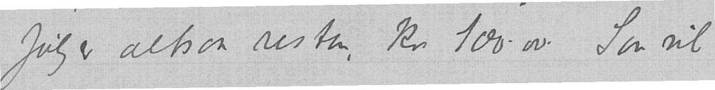følger altsaa resten, kr 100.00. Saa vil
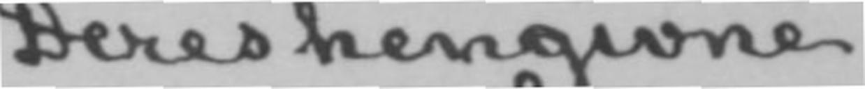Deres hengivne
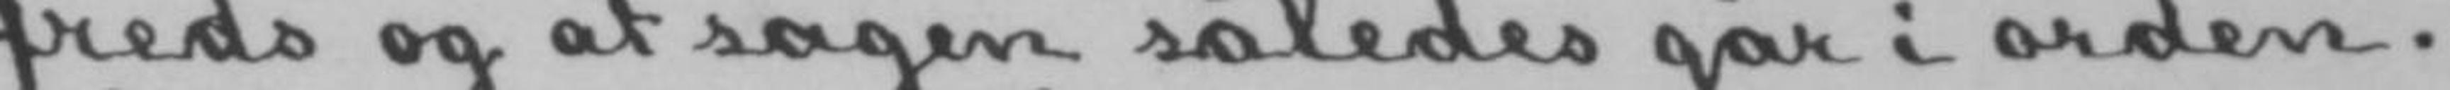freds og at sagen således går i orden.
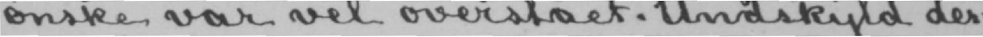ønske var vel overstået. Undskyld der-
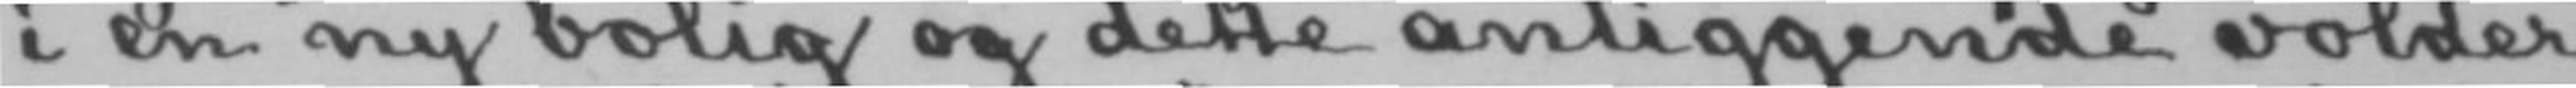i en ny bolig og dette anliggende volder
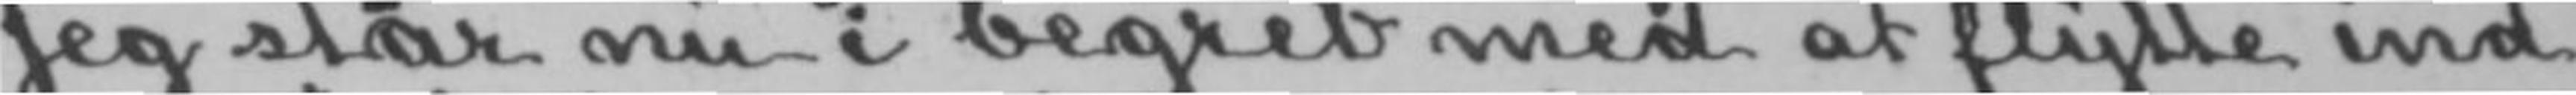Jeg står nu i begreb med at flytte ind
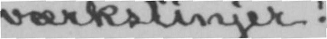værkslinjer!
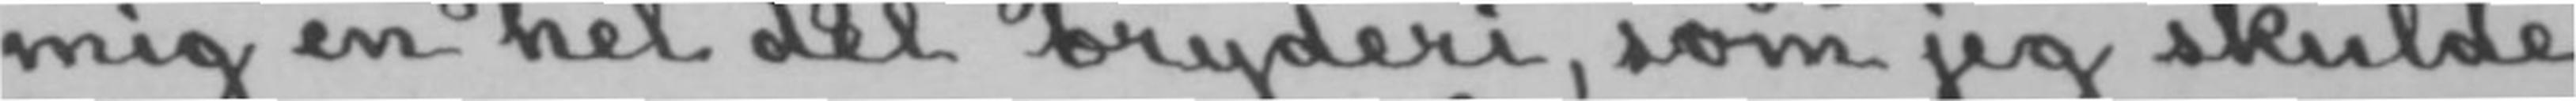mig en hel del bryderi, som jeg skulde
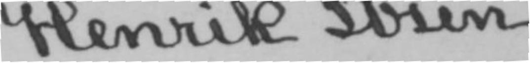Henrik Ibsen.
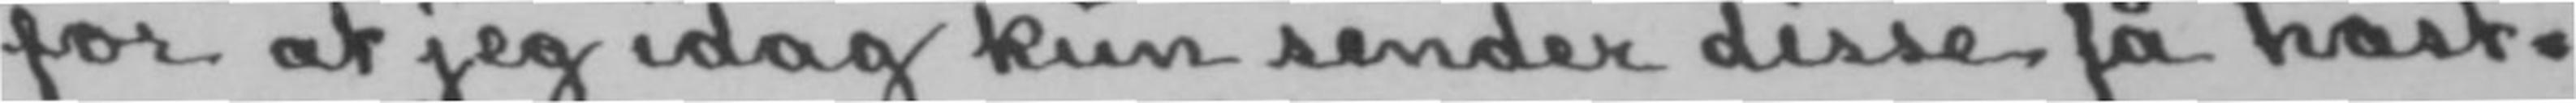for at jeg idag kun sender disse få hast-
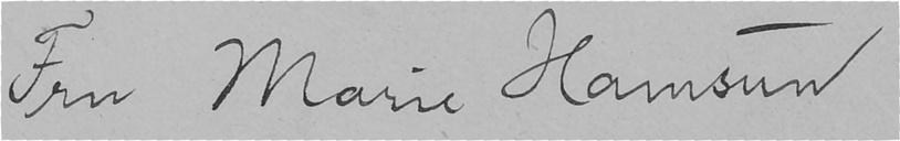Fru Marie Hamsun
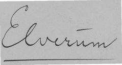Elverum
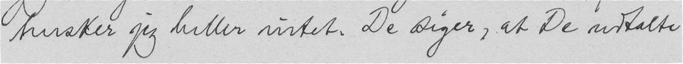husker jeg heller intet. De siger, at De udtalte
